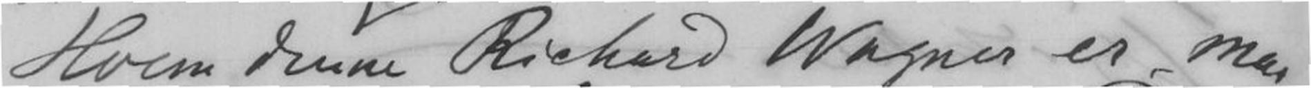Hvem denne Richard Wagner er, maa
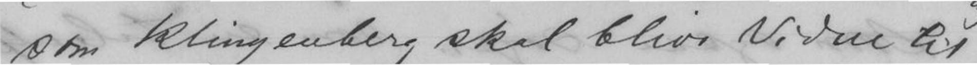som Klingenberg skal blive Vidne til
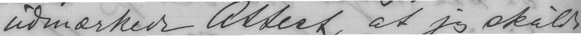udmærkede Attest, at jeg skulde
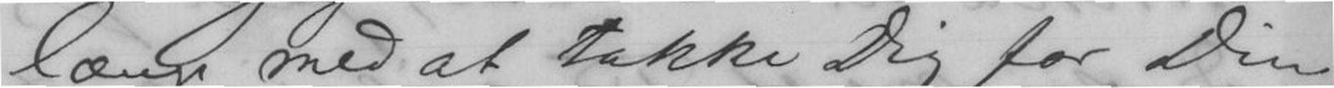længe med at takke Dig for Din
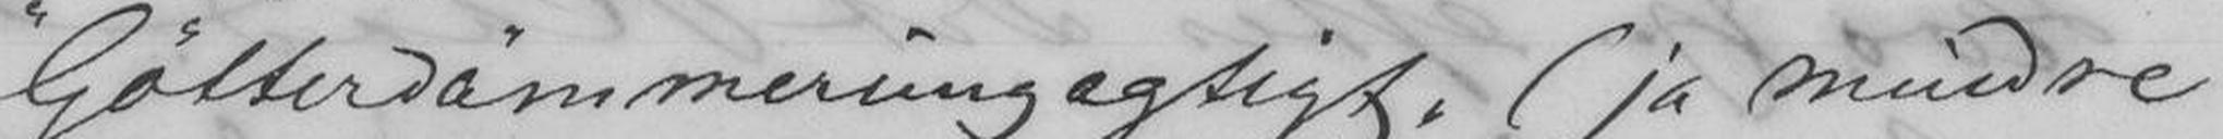"Götterdämmerungagtigt" (ja mindre
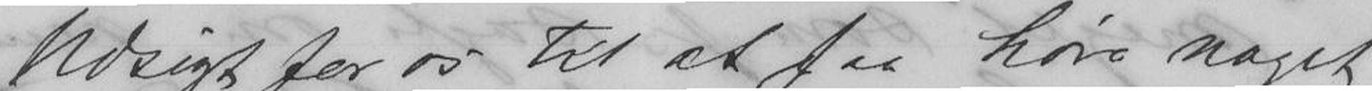Udsigt for os at faa høre noget
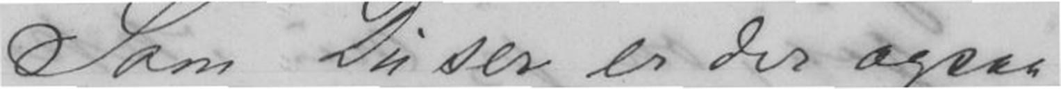Som Du ser er der ogsaa
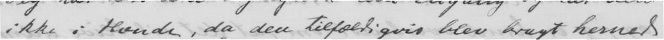ikke i hænde, da den tilfældigvis blev bragt herned
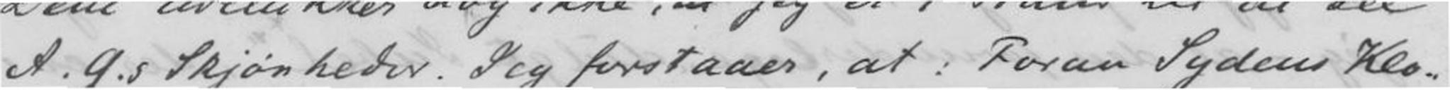A. G.s. Skjønheder. Jeg forstaaer, at: Foran Sydens Klo-
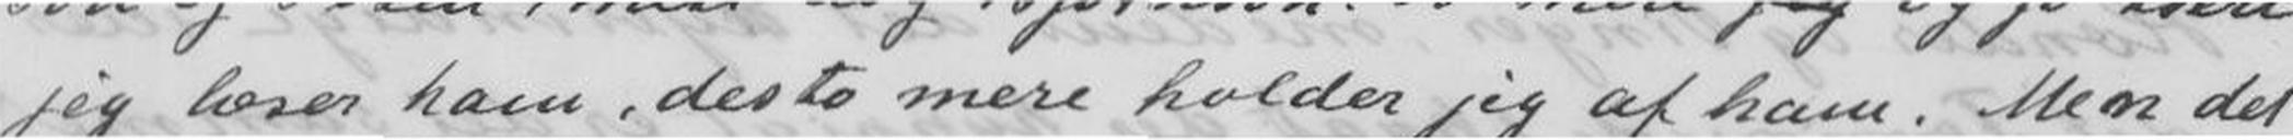jeg læser ham, desto mere holder jeg af ham. Men det
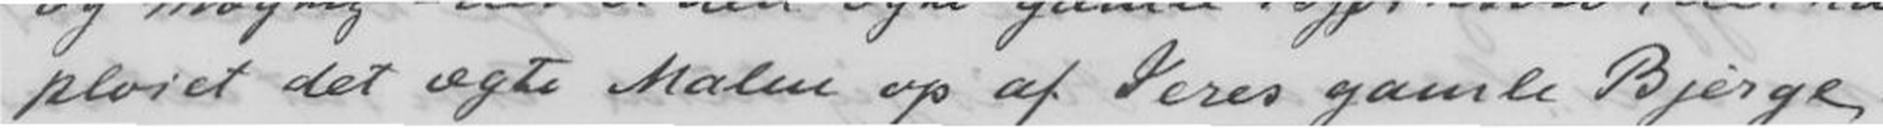ploiet det ægte Malen op af Deres gamle Bjerge.
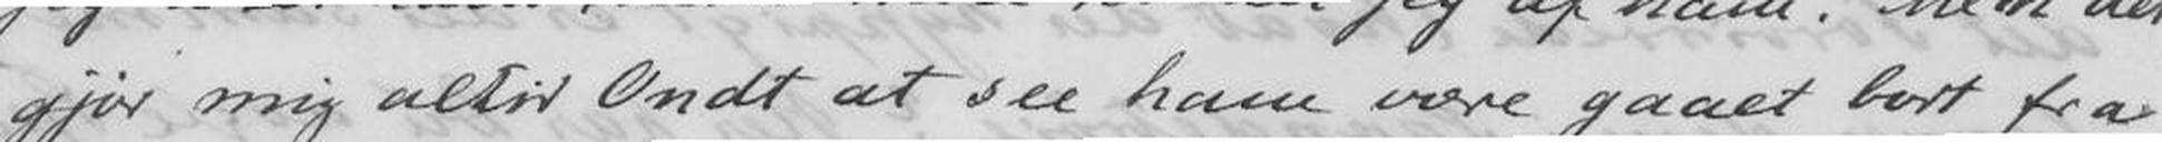gjør mig altid Ondt at see ham være gaaet bort fra
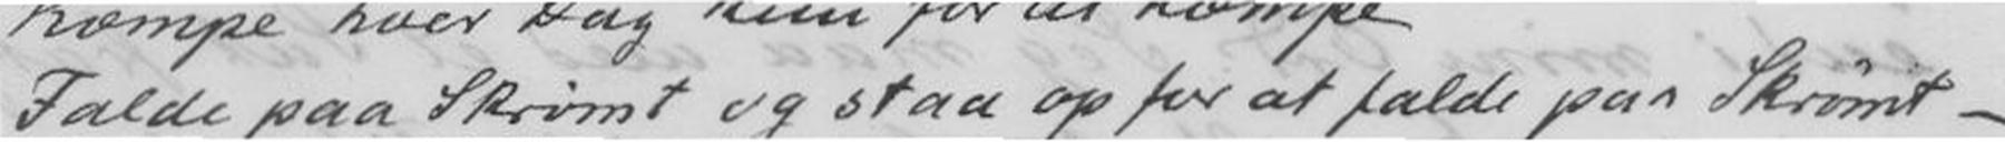Falde paa Skrømt og staa op for at falde paa Skrømt-
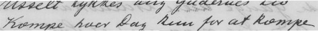Kæmpe hver Dag kun for at Kæmpe
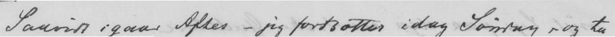Saavidt igaar Aftes - jeg fortsætter idag Sondag, og ta-
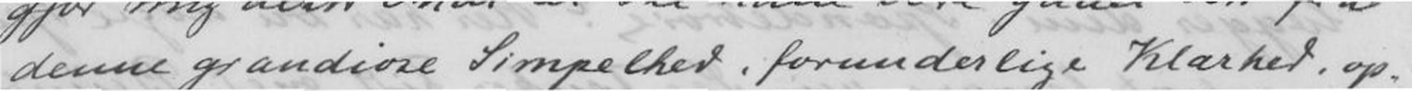denne grandiose Simpelhed, forundelige Klarhed, op-
